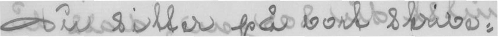Du sitter på vort skrive-
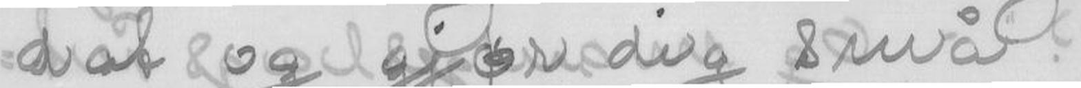dat og gjør dig små
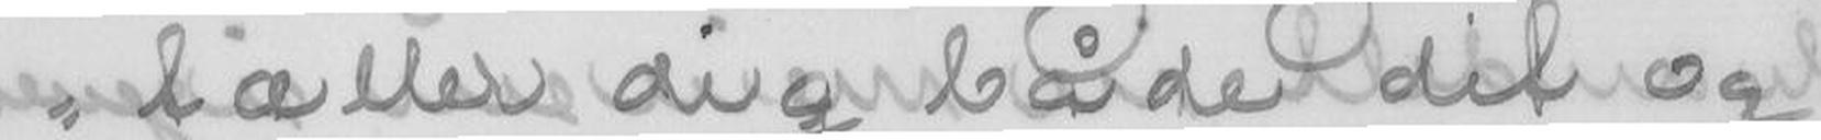tæller dig både dit og
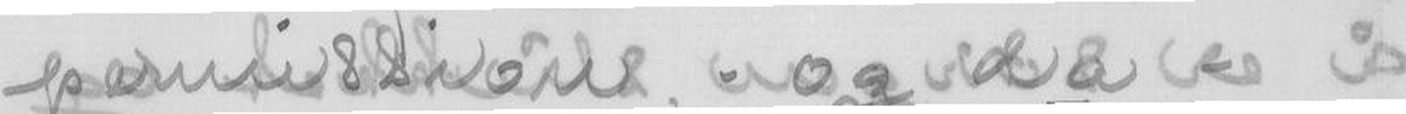permission, - og da -
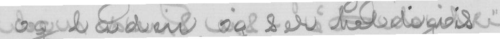og laden, og ser heldigvis
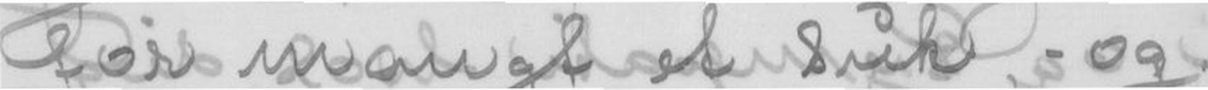før mangt et suk, - og
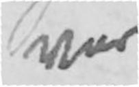var
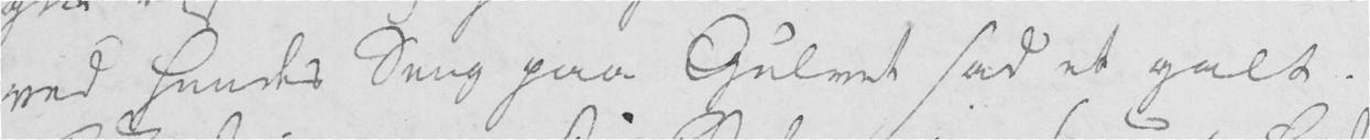ved hendes seng paa Gulvet sad et galt
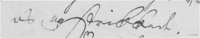os og strikkede. -
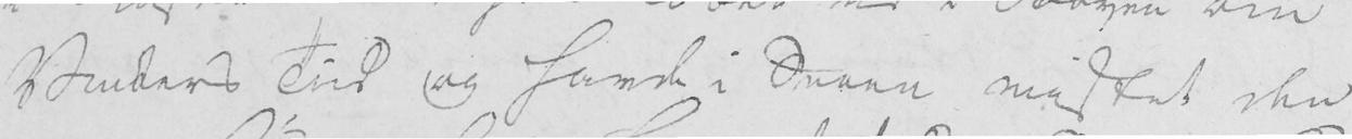Vinters Tiid og havde i Sneen mistet den
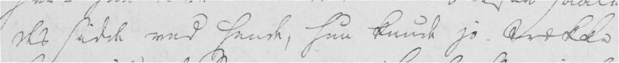des sidde ved hende, hun kunde jo trække
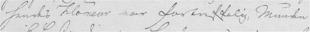hendes Homeur var fortreffelig, Munden
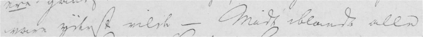vare yderst vilde - Midt iblandt alle
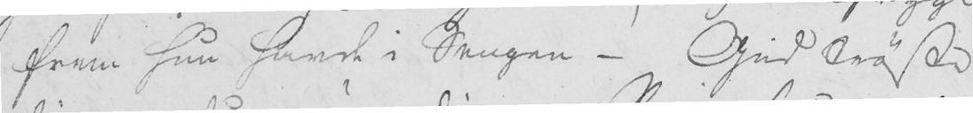frem hun havde i Sengen - Gud trøste
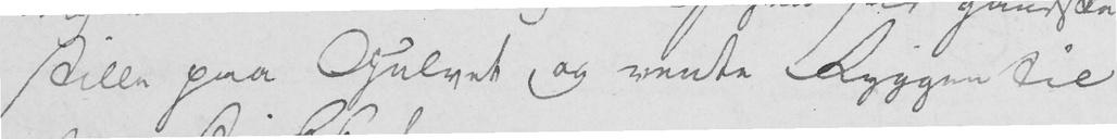stille paa Gulvet og vente Ryggen til
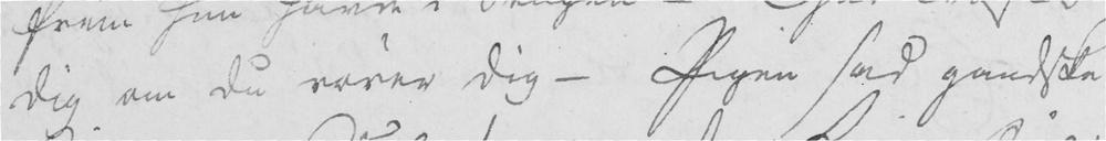dig om du rører dig - Pigen sad gandske
